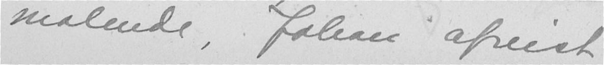malende, Johan afreist
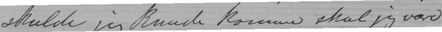skulde jeg kunde komme skal jeg være
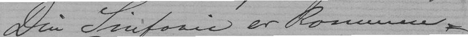Din Sinfoni er kommen
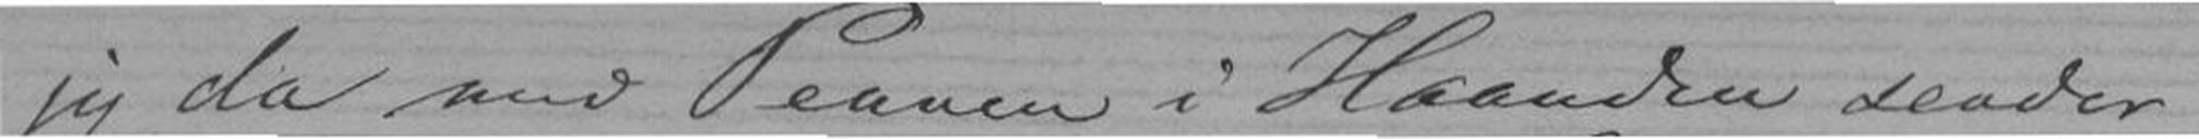jeg da med Pennen i Haanden sender
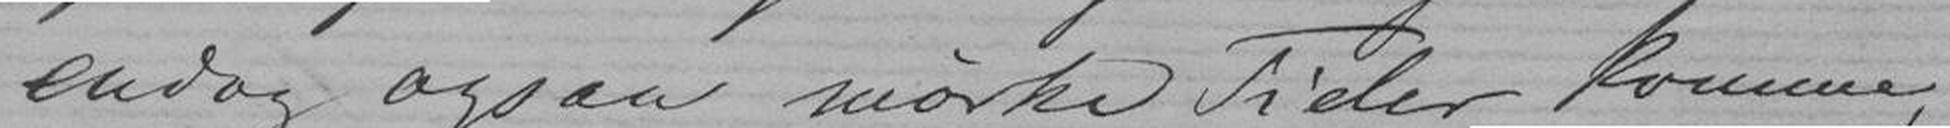endog ogsaa mørke Tider komme,
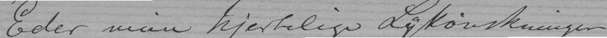Eder mine hjertelige Lykønskninger
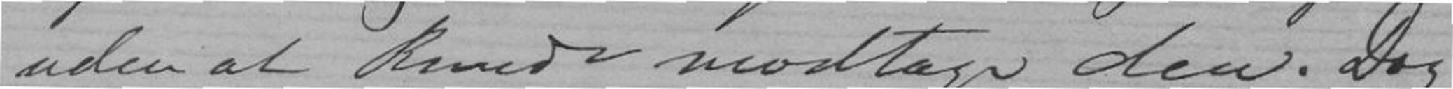uden at kunde modtage den. Dog
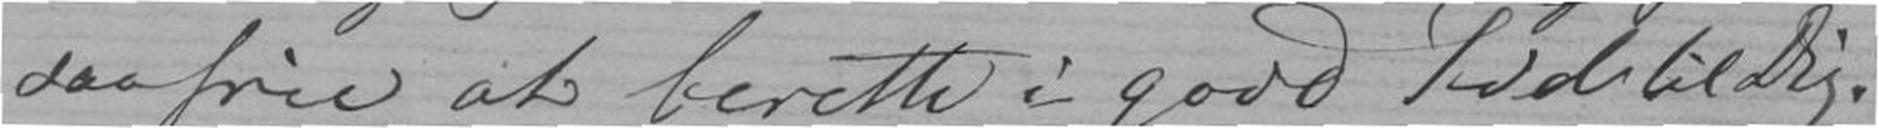saafrie at berette i good Tid til Dig.
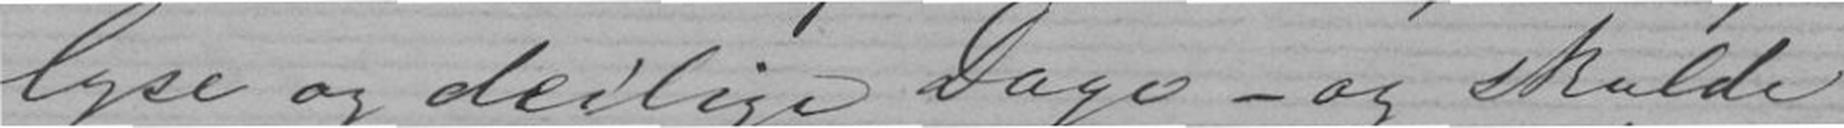lyse og deilige Dage - og skulde
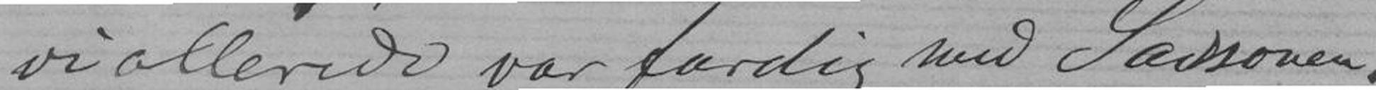vi allerede var færdig med Saisonen.
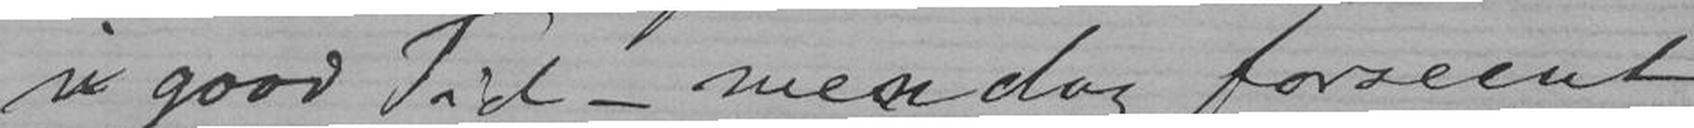i good Tid - men dog forseent
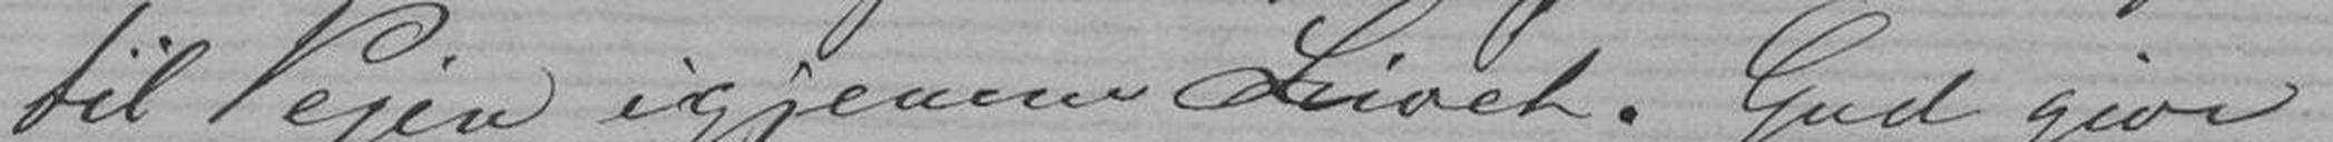til Vejen igjenem Livet. Gud give
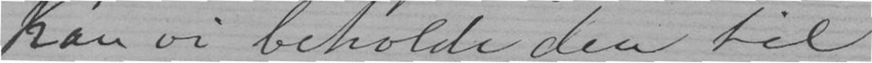Kan vi beholde den til
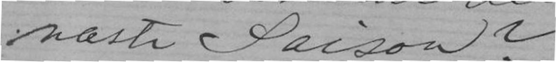næste Saison?
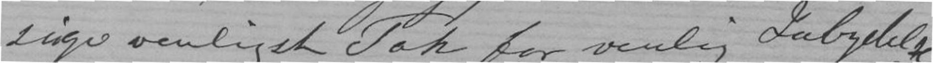sige venligst Tak for venlig Inbydelse
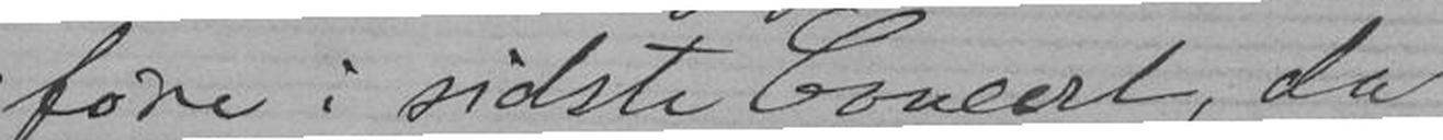føre i sidste Concert, da
